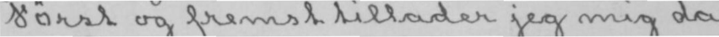Først og fremst tillader jeg mig da
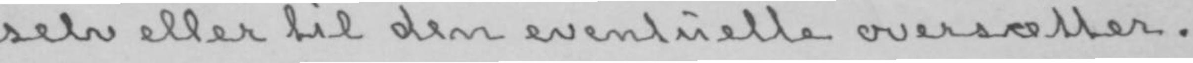selv eller til den eventuelle oversætter.
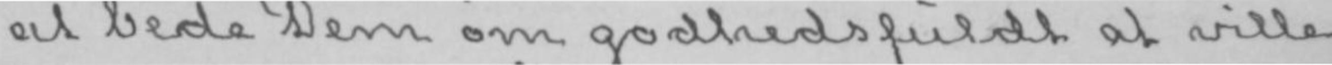at bede Dem om godhedsfuldt at ville
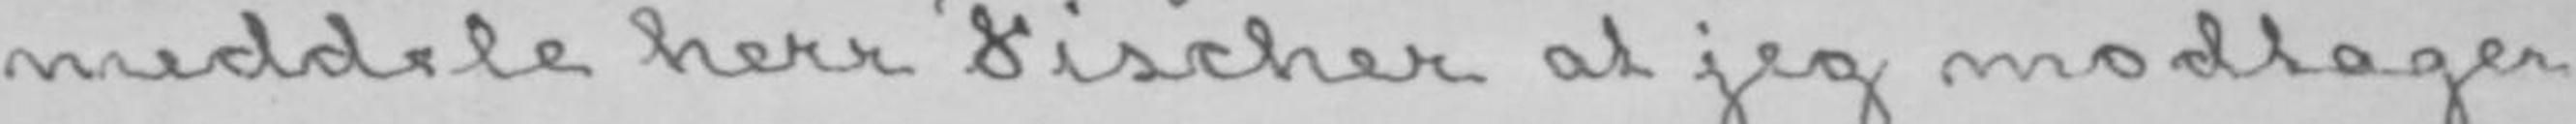meddele herr Fischer at jeg modtager
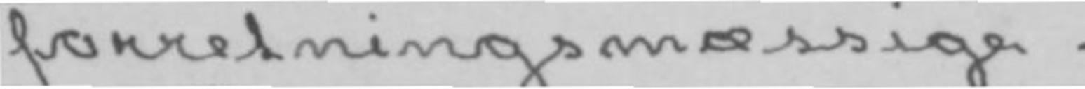forretningsmæssige.
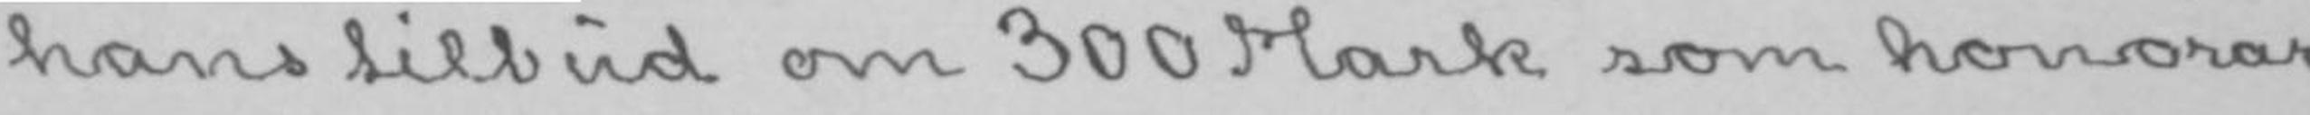hans tilbud om 300 Mark som honorar
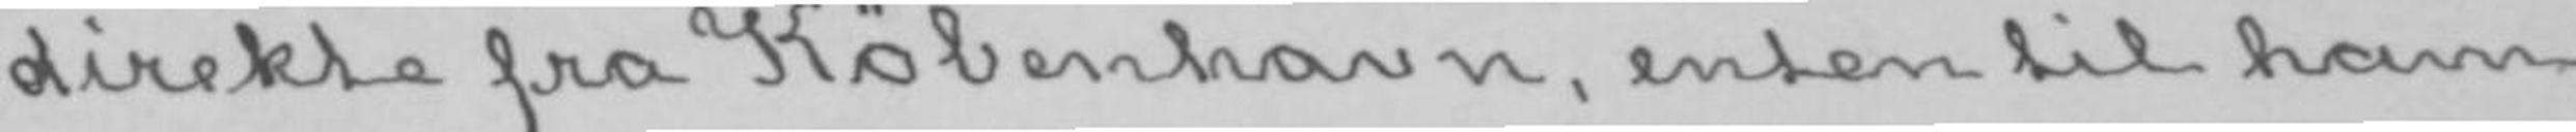direkte fra København, enten til ham
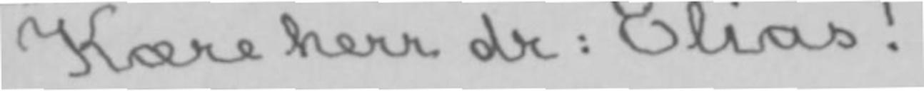Kære herr dr: Elias!
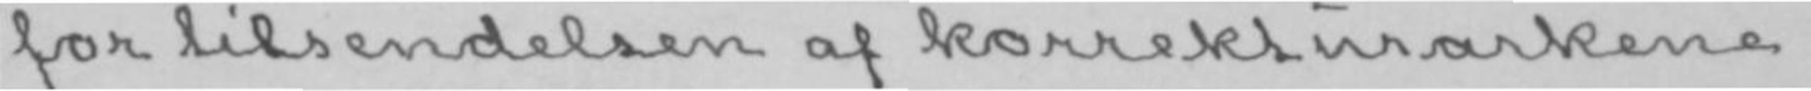for tilsendelsen af korrekturarkene
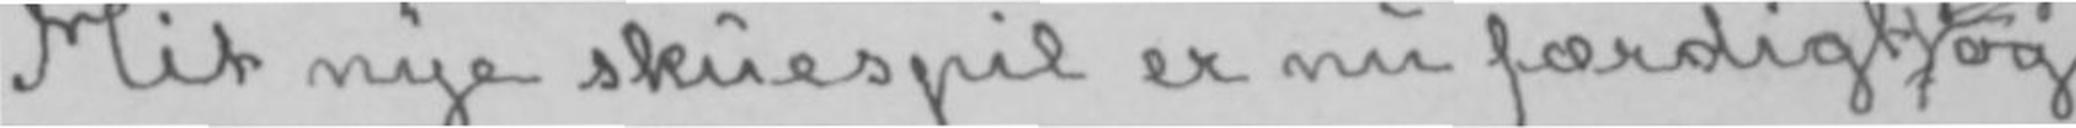Mit nye skuespil er nu færdigt jeg og
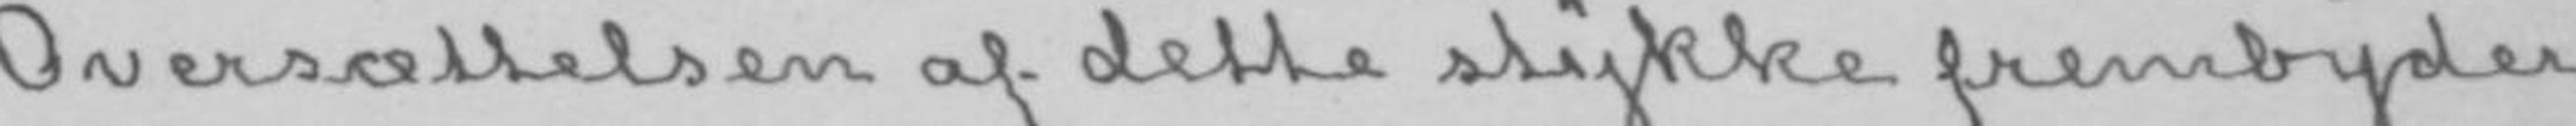Oversættelsen af dette stykke frembyder
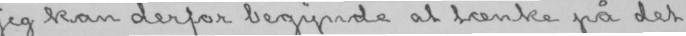jeg kan derfor begynde at tænke på det
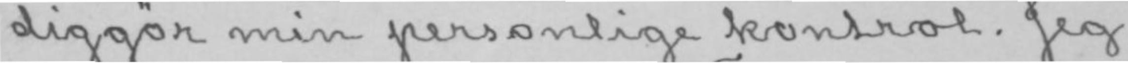diggør min personlige kontrol. Jeg
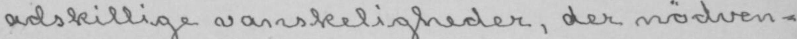adskillige vanskeligheder, der nødven-
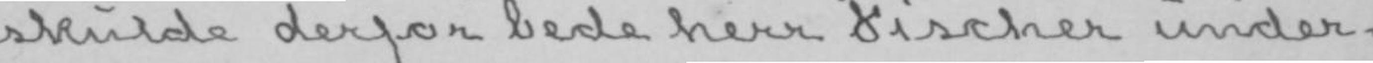skulde derfor bede herr Fischer under-
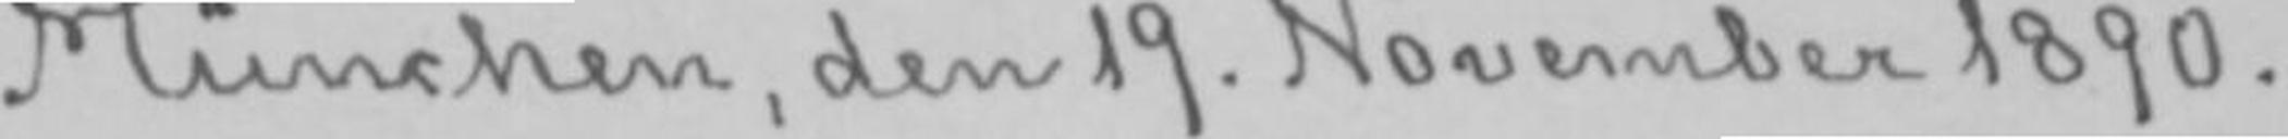München, den 19. November 1890.
